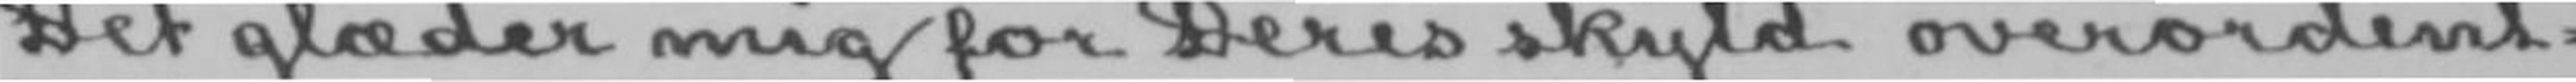Det glæder mig for Deres skyld overordent-
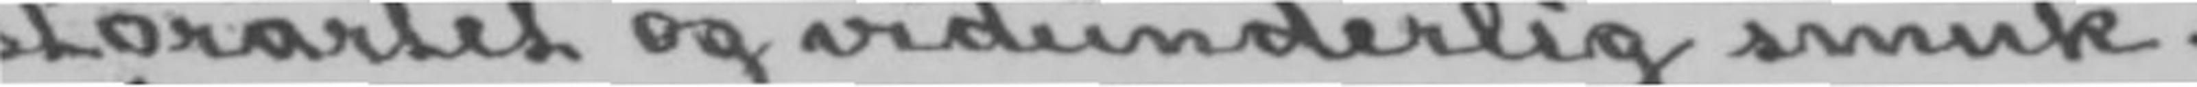storartet og vidunderlig smuk.
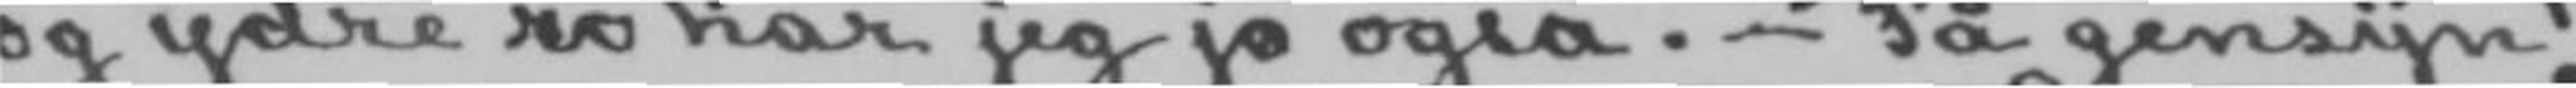og ydre ro har jeg jo også.- På gensyn!
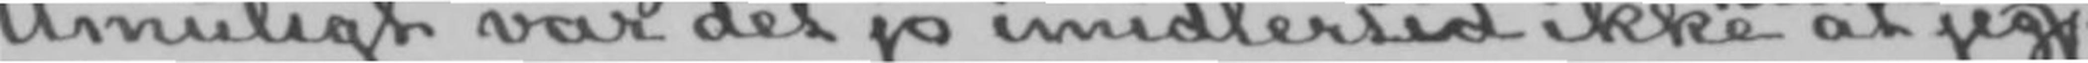Umuligt var det jo imidlertid ikke at jeg
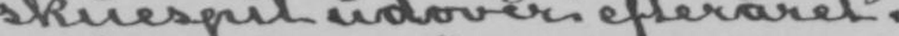skuespil udover efteråret.
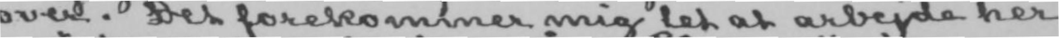over. Det forekommer mig let at arbejde her
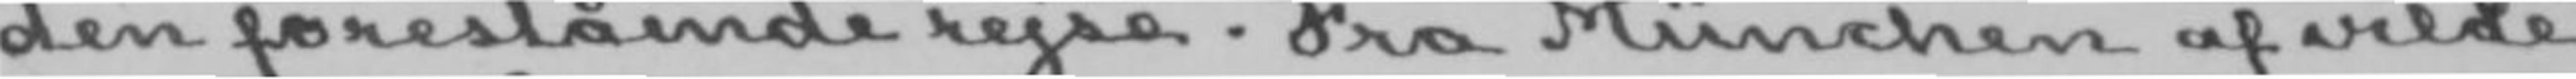den forestående rejse. Fra München af vilde
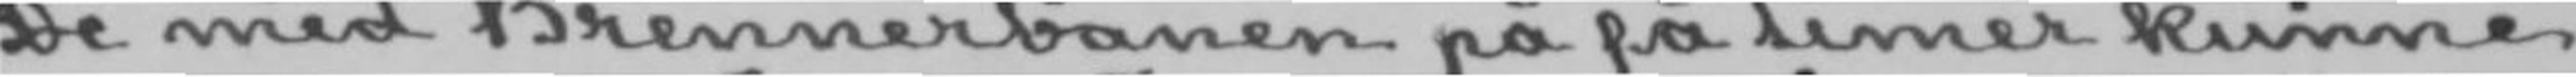De med Brennerbanen på få timer kunne
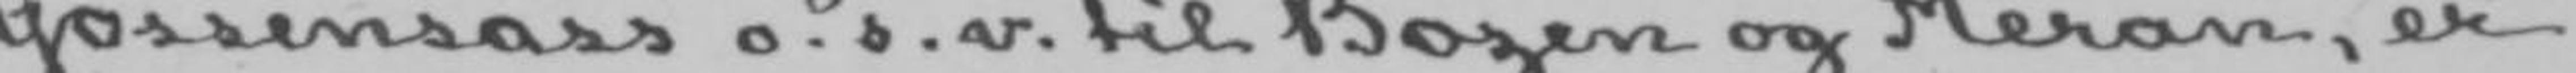Gossensass o. s. v. til Bozen og Meran, er
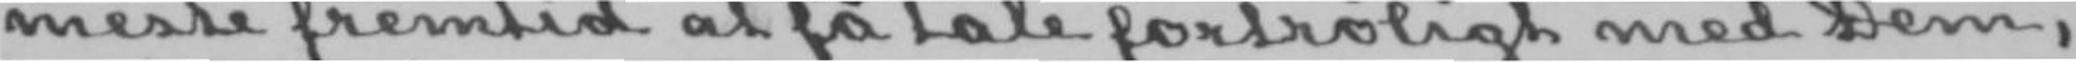meste fremtid at få tale fortroligt med Dem,
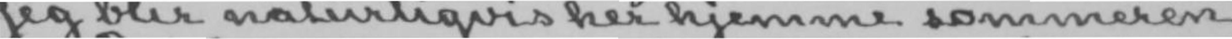Jeg blir naturligvis her hjemme sommeren
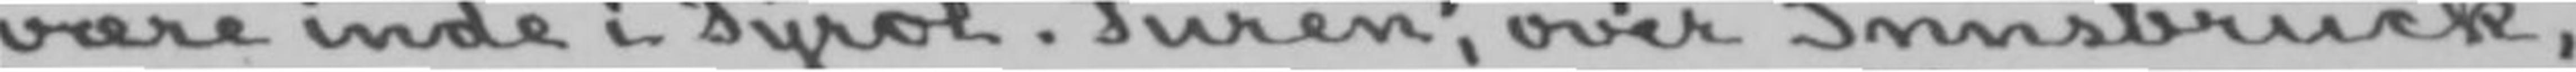være inde i Tyrol. Turen, over Innsbruck,
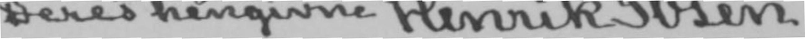Deres hengivne Henrik Ibsen.
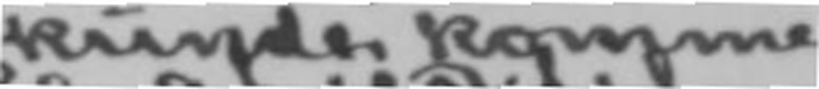kunde komme
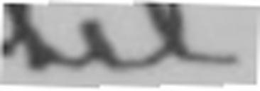til
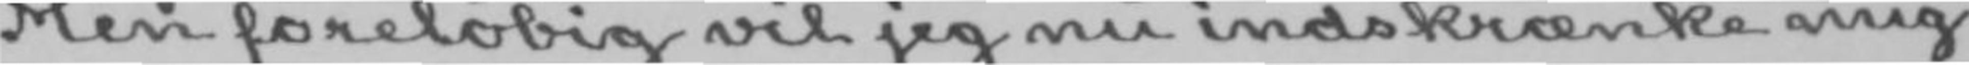Men foreløbig vil jeg nu indskrænke mig
